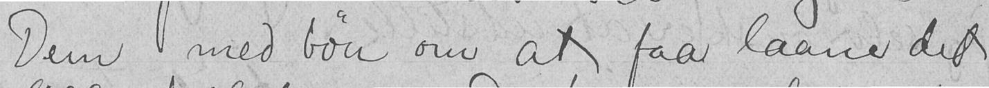Dem med bøn om at faa laane det
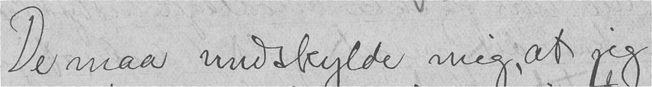De maa undskylde mig, at jeg
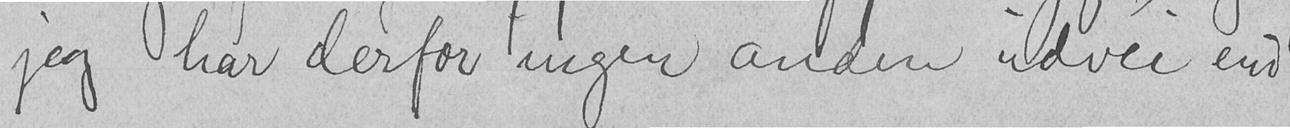jeg har derfor ingen anden udvei end
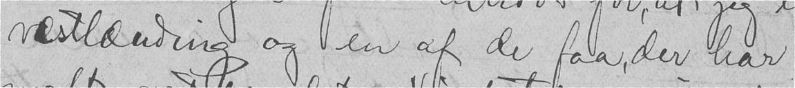væstlænding og en af de faa, der har
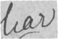har
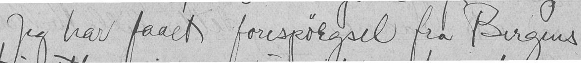Jeg har faaet forespørgsel fra Bergens
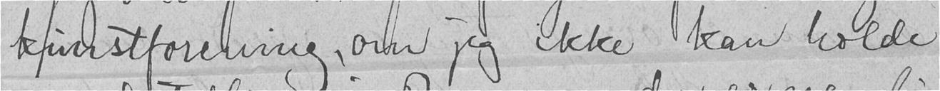kunstforening, om jeg ikke kan holde
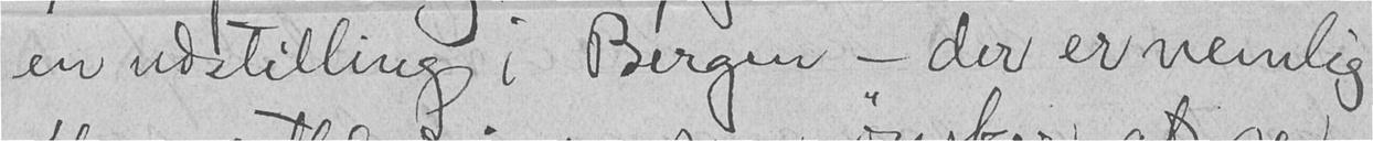en udstilling i Bergen - der er nemlig
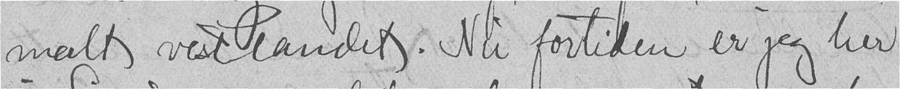malt vestlandet. Nu fortiden er jeg her
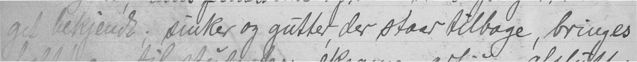get bekjendt; sinker og gutter, der staar tilbage, bringes
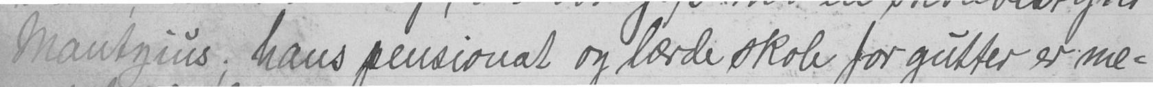Mantyius; hans pensionat og lærde skole for gutter er me-
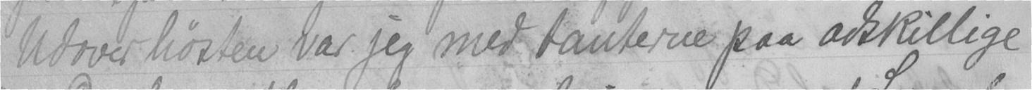Udover høsten var jeg med tanterne paa adskillige
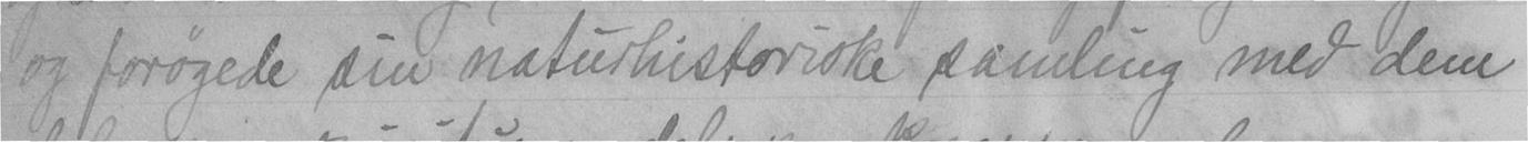og forøgede sin naturhistoriske samling med dem
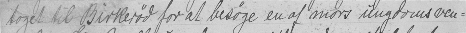toget til Birkerød for at besøge en af mors ungdomsven-
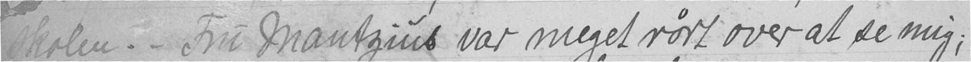skolen. - Fru Mantzius var meget rørt over at se mig;
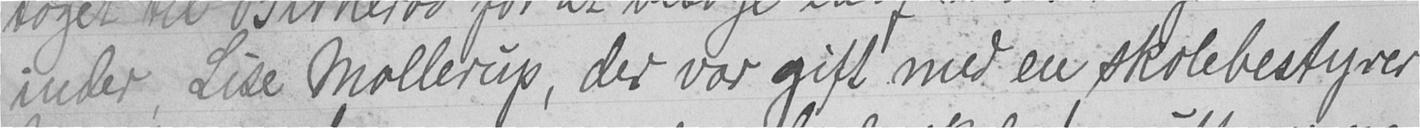inder, Lise Mollerup, der var gift med en skolebestyrer
